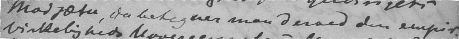Modsætn, da betegner man derved den empir.
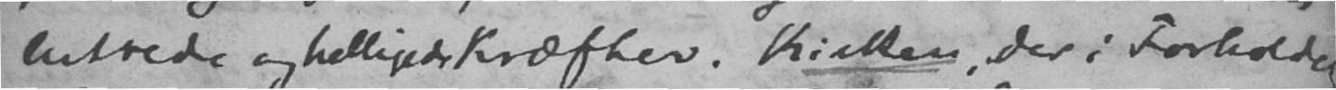lutrede og hellige Kræfter. Kirken, der i Forholdet
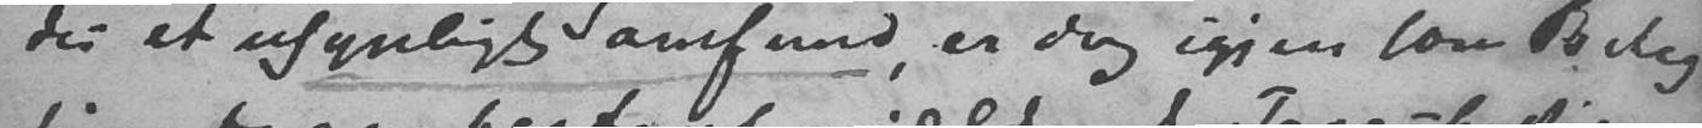des et usynligt Samfund, er dog igjen som Betyd-
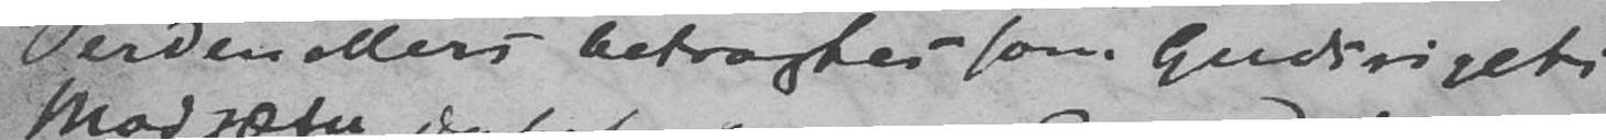Verden ellers betragtes som Gudsrigets
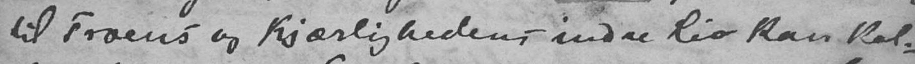til Troens og Kjærlighedens indre Liv kan kal-
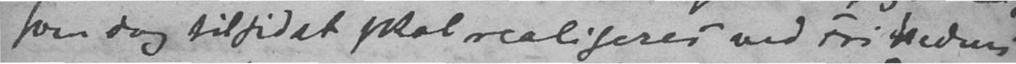som dog tilsidst skal realiserer ved Frihedens
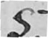C.
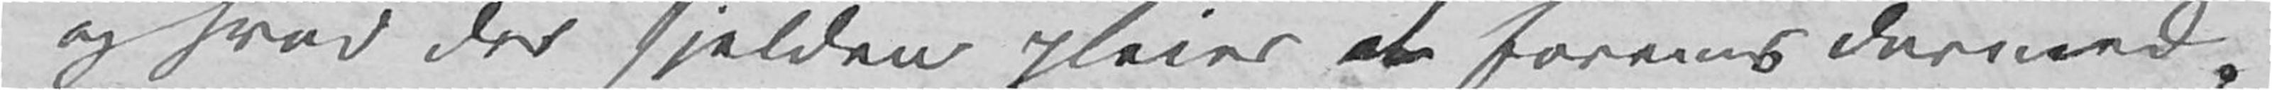og hvad der sjelden pleier at forenes dermed,
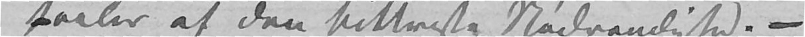taaler af den bittreste Nødvendighed. –
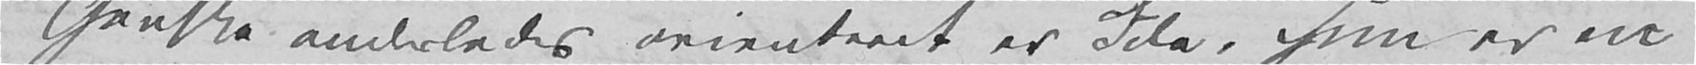Ganske anderledes orienteret er Ida, hun er en
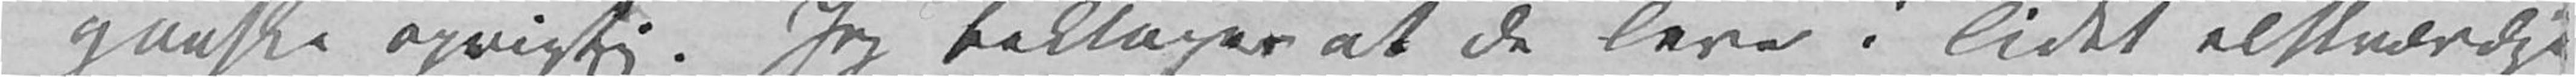ganske oprigtig. Jeg beklager at de leve i lidet elskværdige
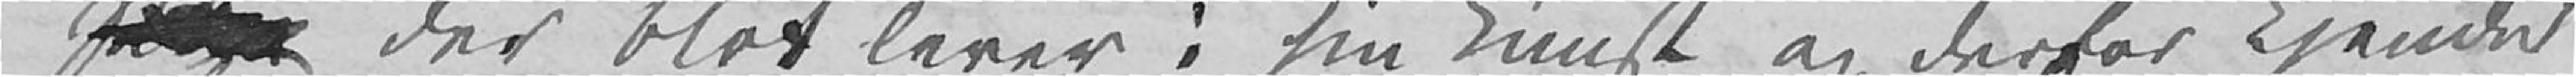Pige der blot lever i sin Kunst og derfor kjender
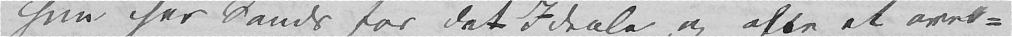hun har Sands for det Ideale og ofte et over¬
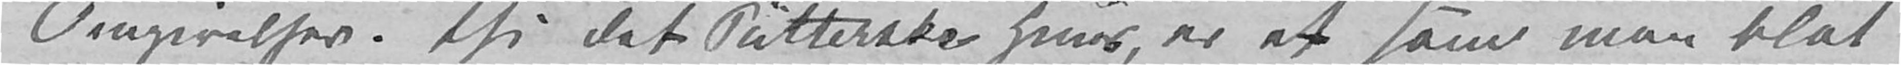Omgivelser, thi det Pütterske Huus, er et som man blot
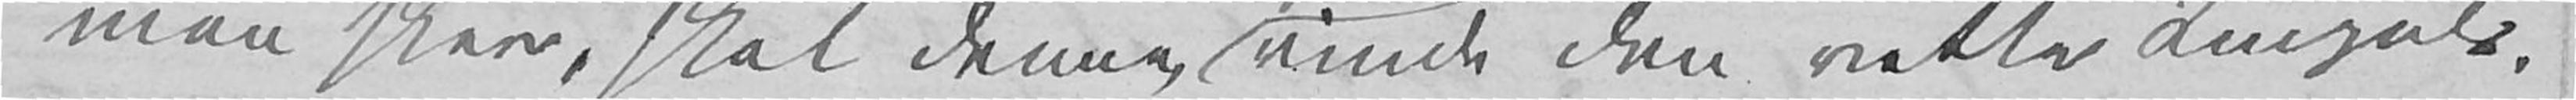maa skee, skal denne vinde den rette Impuls.
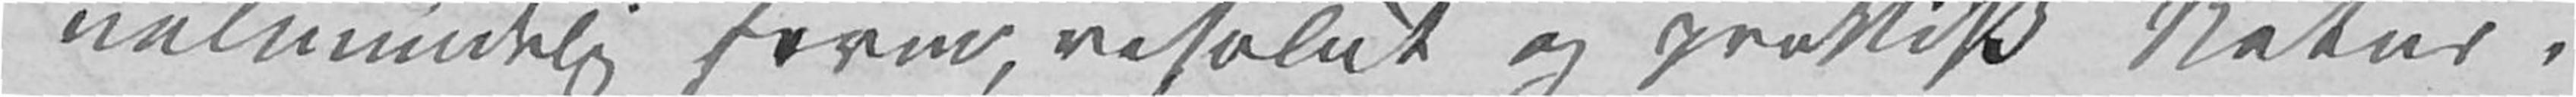ualmindelig ferm, resolut og praktisk Natur,
